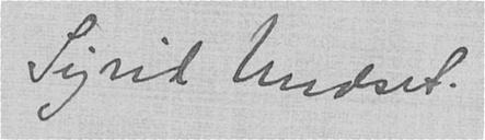Sigrid Undset.
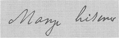Mange hilsener
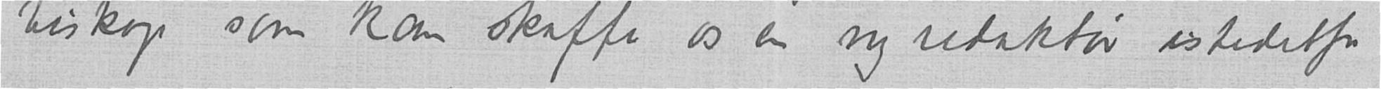biskop som kan skaffe os en ny redaktør istedetfor
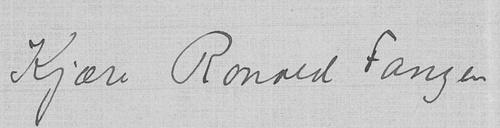Kjære Ronald Fangen
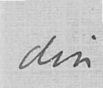din
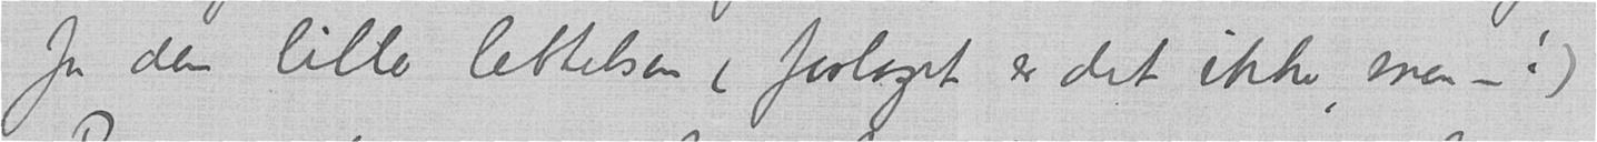for den lille lettelsen (forlaget er det ikke, men -!)
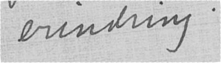erindring.
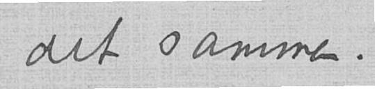det sammen.
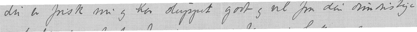du er frisk nu og har sluppet godt og vel fra din dumdristige
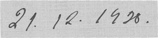21.12.1928.
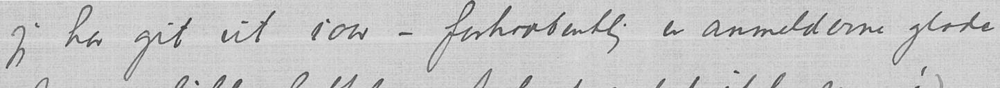jeg har git ut iaar – forhaabentlig er anmelderne glade
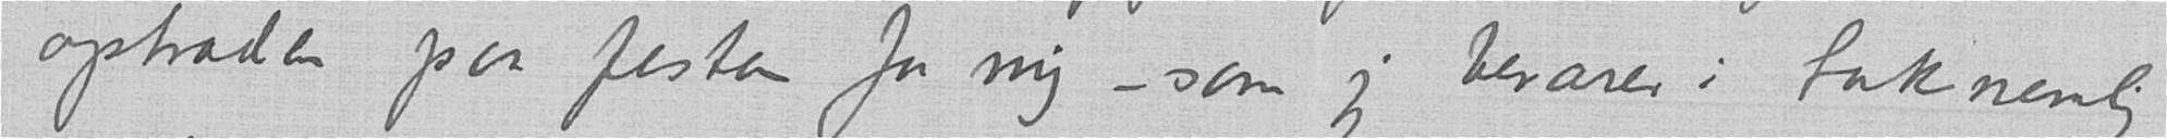optræden paa festen for mig – som jeg bevarer i taknemlig
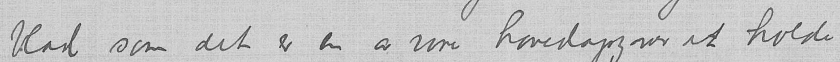blad som det er en av vore hovedopgaver at holde
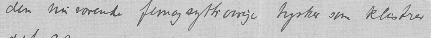den nu værende femogsyttiaarige tysker som klistrer
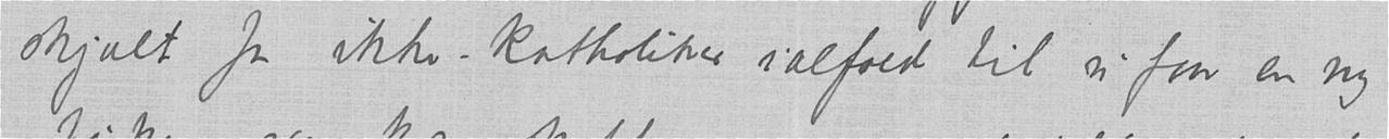skjult for ikke-katholiker ialfald til vi faar en ny
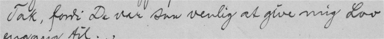Tak, fordi De var saa venlig at give mig Lov
